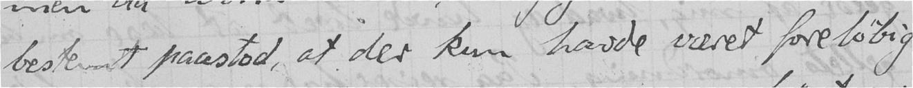bestemt paastod, at der kun havde været foreløbig
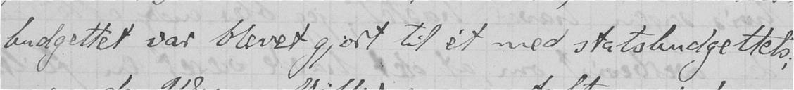budgettet var blevet gjort til ét med statsbudgettets;
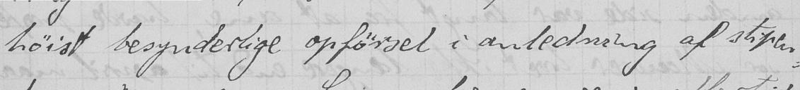høist besynderlige opførsel i anledning af stipen-
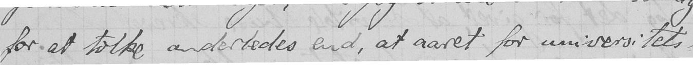for at tolke anderledes end, at aaret for universitets-
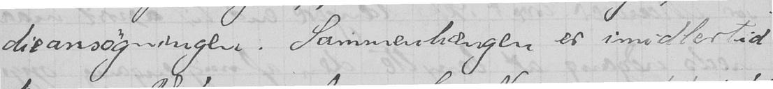dieansøgningen. Sammenhængen er imidlertid
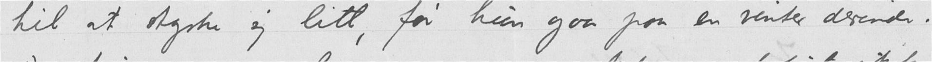til at styrke sig litt, før hun gaar paa en vinter derinde.
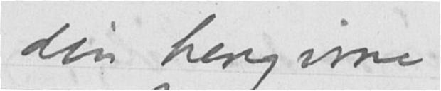din hengivne
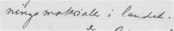ningsmaterialer i landet.
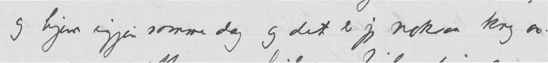og hjem igjen samme dag og det er jeg noksaa kry av.
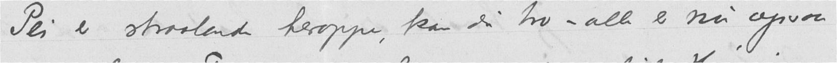Pei er straalende heroppe, kan du tro – alle er nu ogsaa
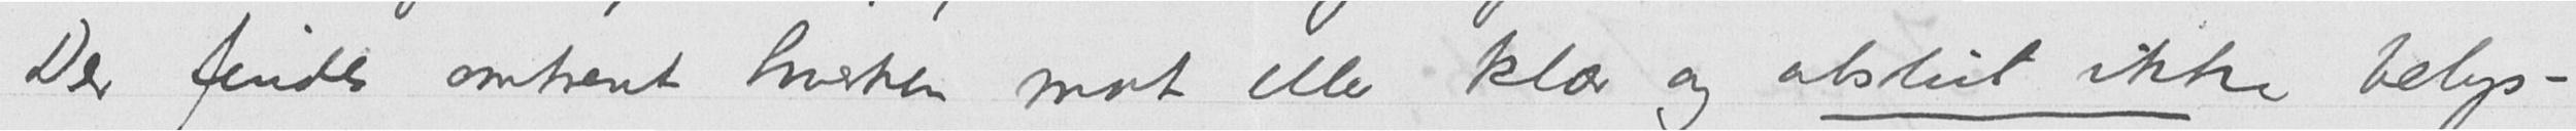Der findes omtrent hverken mat eller klær og absolut ikke belys-
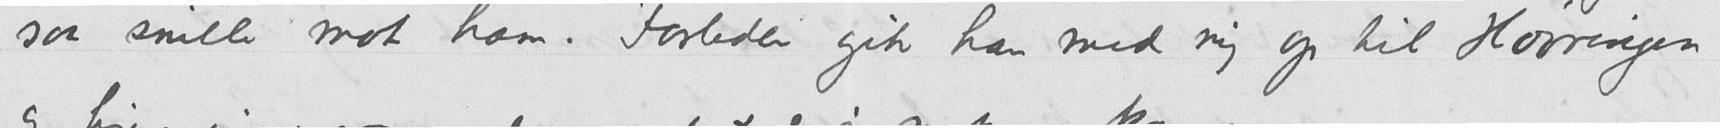saa snille mot ham. Forleden gik han med mig op til Høvringen
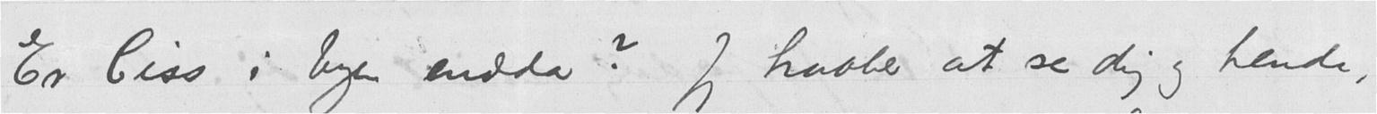Er Ciss i byen endda? Jeg haaber at se dig og hende,
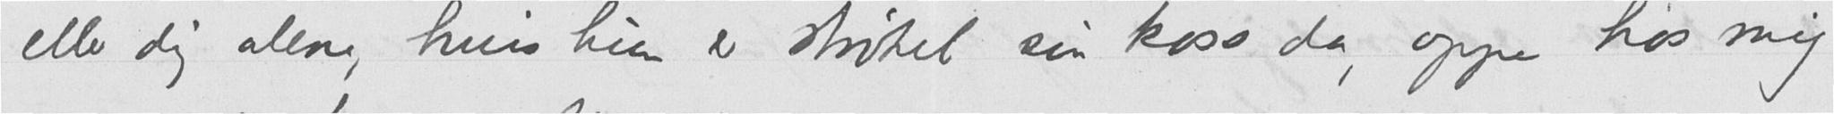eller dig alene, hvis hun er strøket sin koss da, oppe hos mig
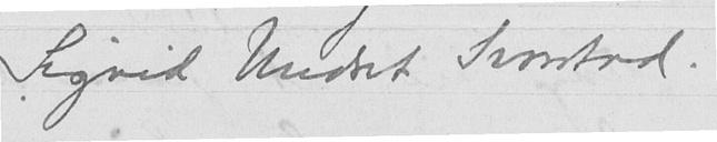Sigrid Undset Svarstad.
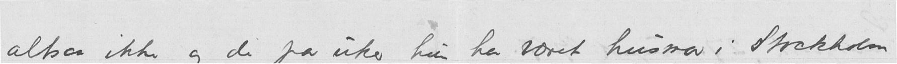altsaa ikke og de par uke hun har været husmor i Stockholm
